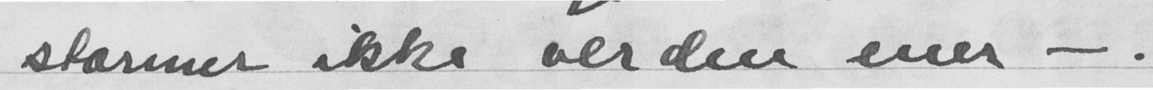stormer ikke verden mer - .
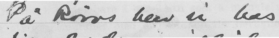På Røros blev vi hos
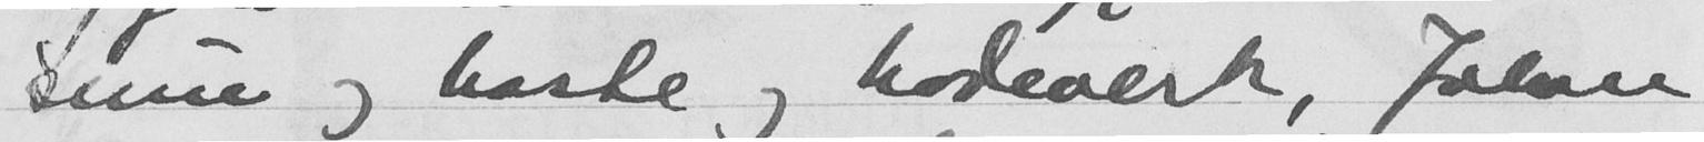Snue og hoste og hodeverk, Johan
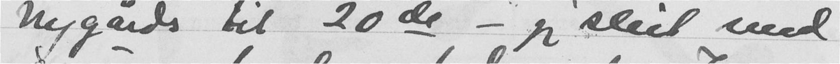Nygårds til 20de - jeg sleit med
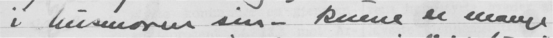i husmoren sin - kuene er mange
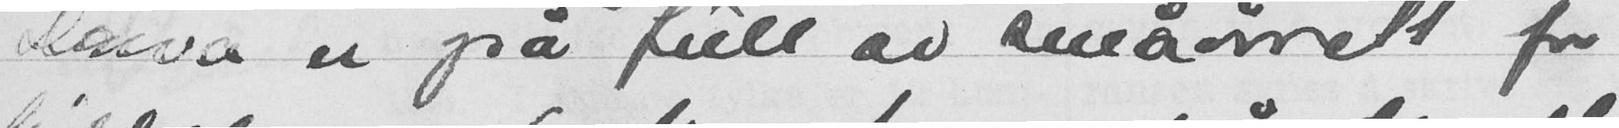laa er også full av småørrett for
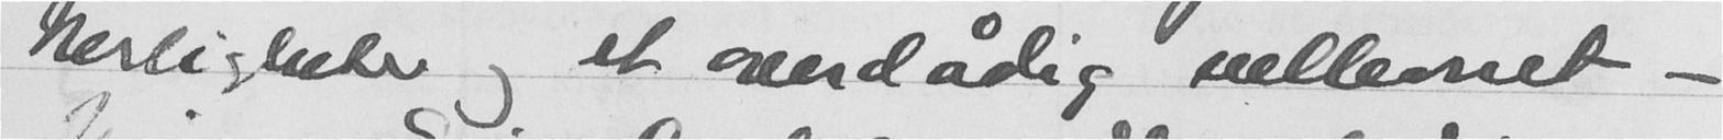herligheter og et overdådig vellevnet -
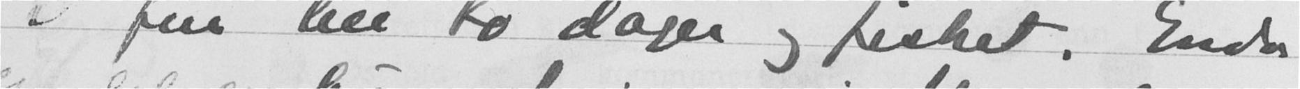i rie lle to dager og fisket. Enda
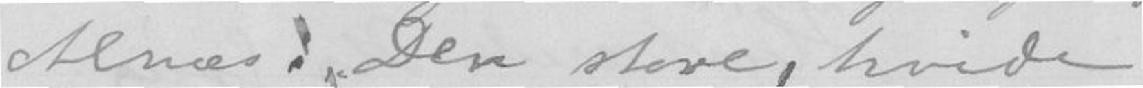Alnæs. "Den store, hvide
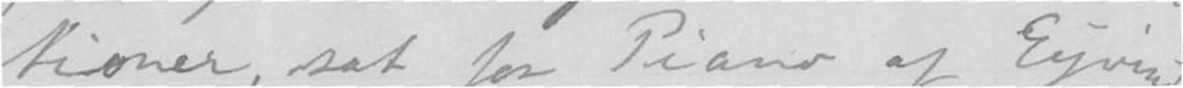tioner, sat for Piano af Eyvind
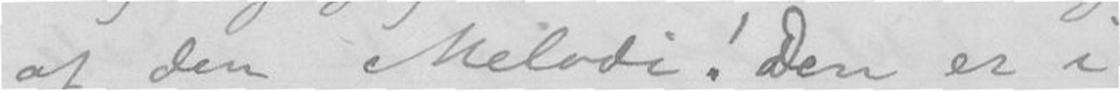af den Melodi! Den er i
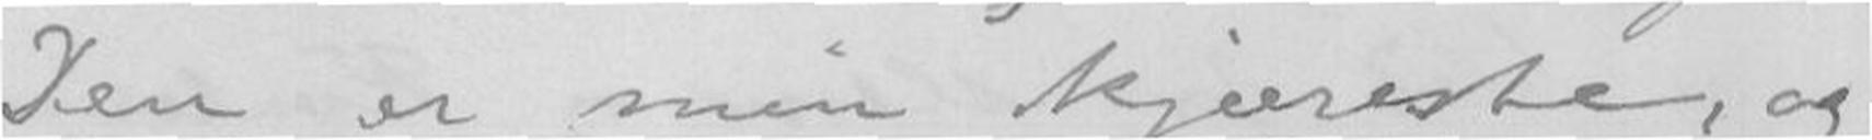Den er min kjæreste, og
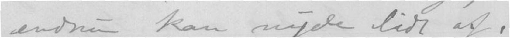endnu kan nyde lidt af.
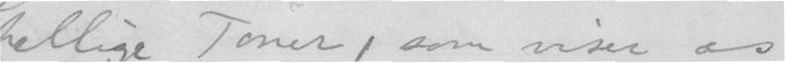hellige Toner, som viser os
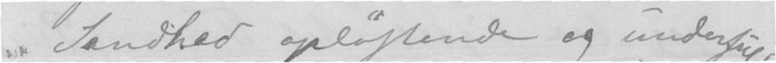Sandhed opløftende og underfuld
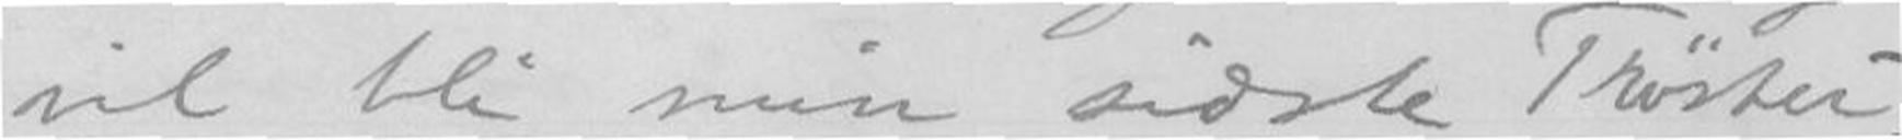vil bli min sidste Trøster.
